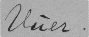Vuer.
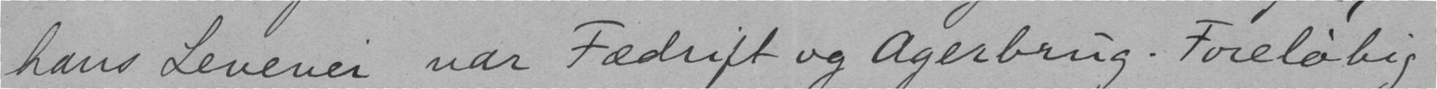hans Levevei var Fædrift og Agerbrug. Foreløbig
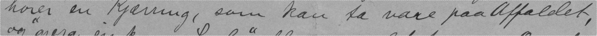hører en Kjærring, som kan ta vare paa Affaldet,
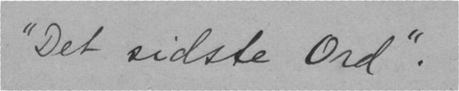"Det sidste Ord".
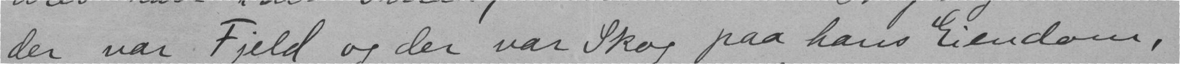der var Fjeld og der var Skog paa hans Eiendom,
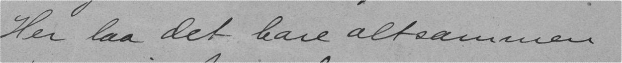Her laa det bare altsammen
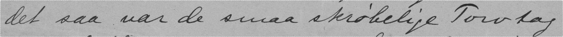det saa var de smaa skrøbelige Torvtag
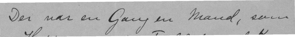Der var en Gang en Mand, som
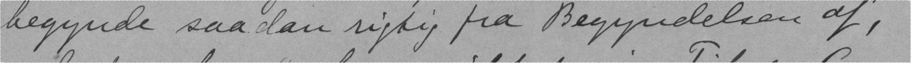begynde saadan rigtig fra Begyndelsen af,
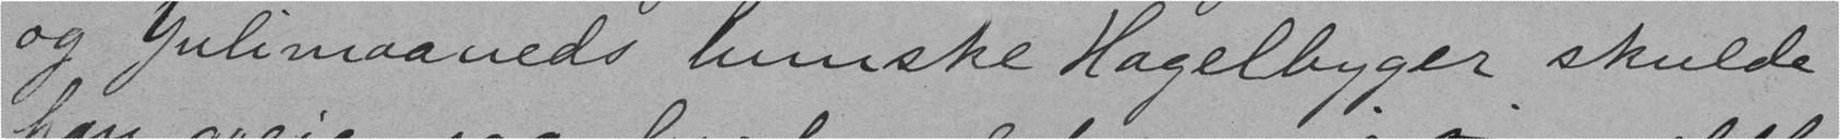og Julimaaneds lumske Hagelbyger skulde
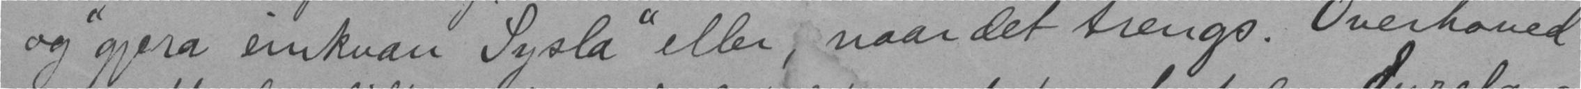og "gjera einkvar Sysla" eller, naar det trengs. Overhoved
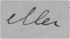eller
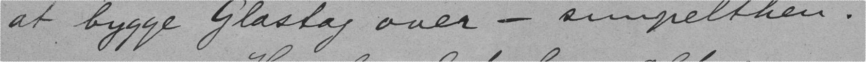at bygge Glastag over - simpelthen.
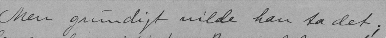Men grundigt vilde han ta det;
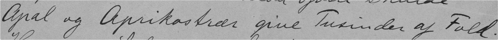Apal og Aprikostrær give Tusinder af Fold.
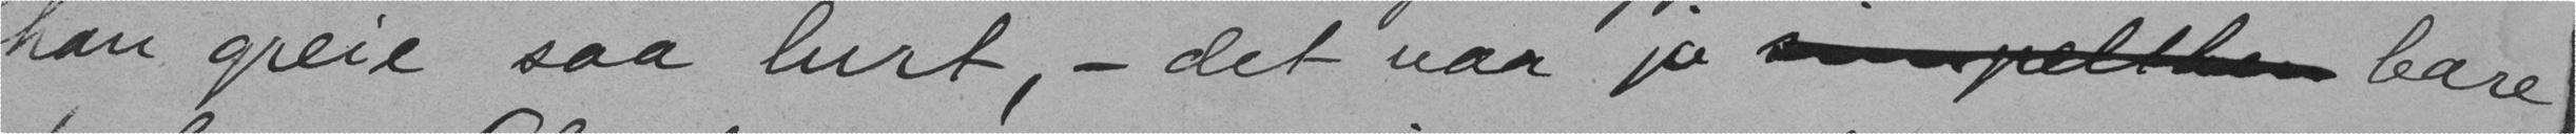han greie saa lurt, - det var jo simpelthen bare
